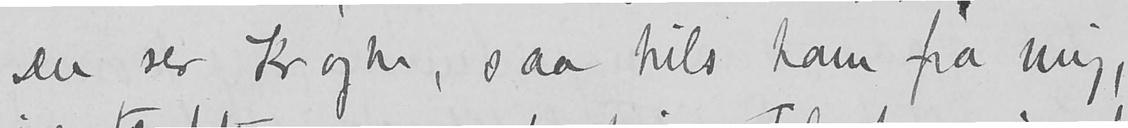Du ser Krogh, saa hils ham fra mig,
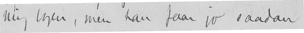ning bogen, men han faar jo saadan
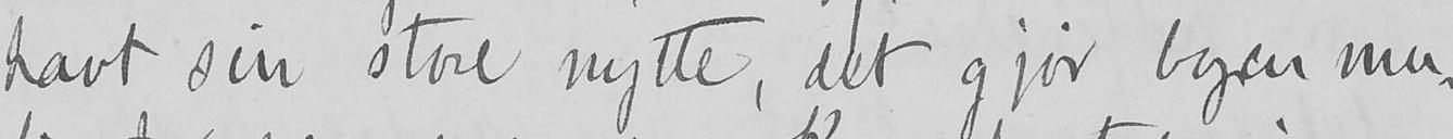havt sin store nytte, det gjør bogen mu-
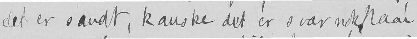det er sandt, kanske det er svar nok. Naar
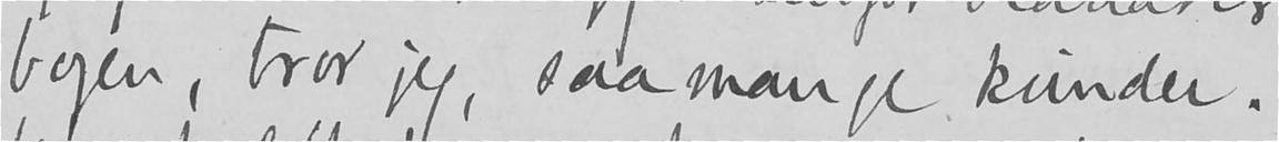bogen, tror jeg, saa mange kvinder.
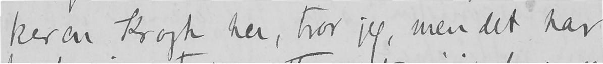keren Krogh her, tror jeg, men det har
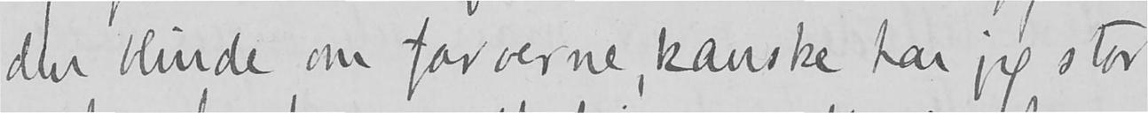den blinde om farverne, kanske har jeg stor
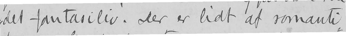det fantasiliv. Der er lidt af romanti-
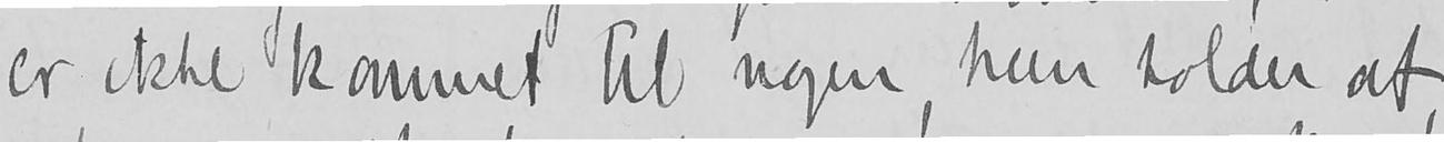er ikke kommet til nogen, hun holder af,
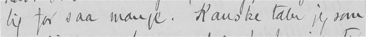lig for saa mange. Kanske taler jeg som
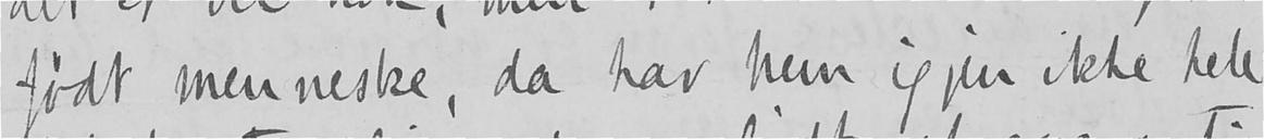født menneske, da har hun igjen ikke hele
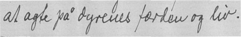at agte på dyrenes færden og liv.
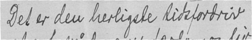Det er den herligste tidsfordriv.
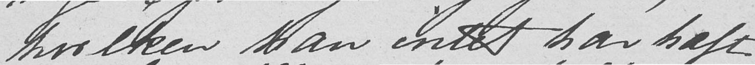hvilken han intet har haft
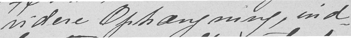videre Ophængning, ind-
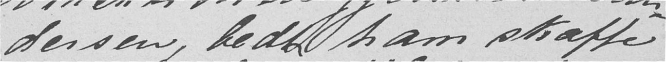dersen, bedt ham skaffe
